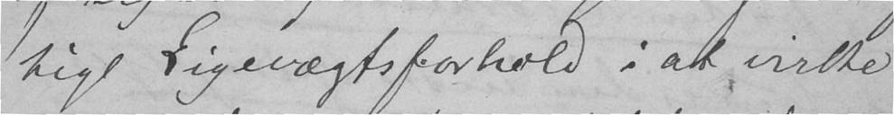tige Ligevægtsforhold: at virke
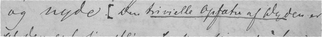og nyde [Den trivielle Opfatn af Dyden er
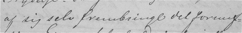af sig selv frembringe det fornuf-
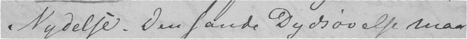Nydelse. Den sande Dydsøvelse maa
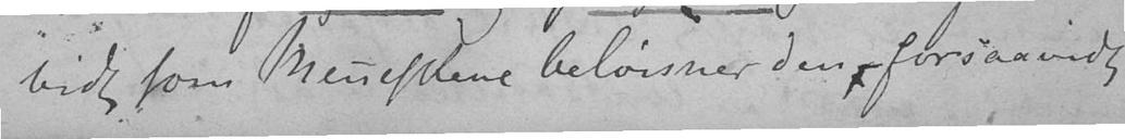vidt som Menneskene belønner den forsaavidt
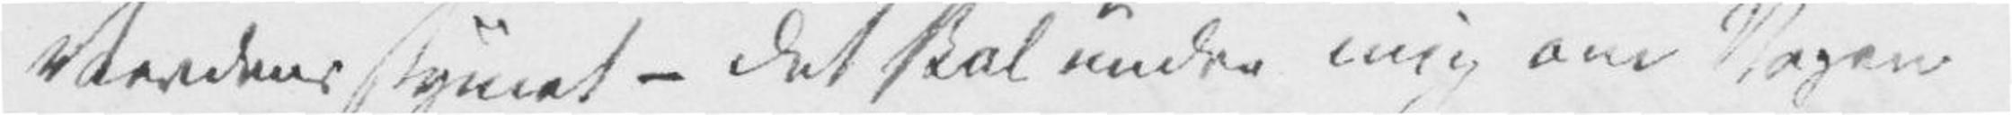Verdensstmet – det skal undre mig om Nogen
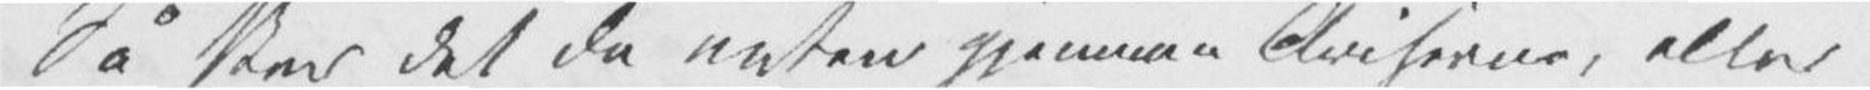Så sker det da enten gjennem Aviserne, eller
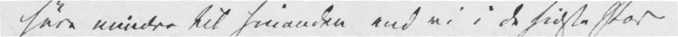høre mindre til hinanden end vi i de sidste Par
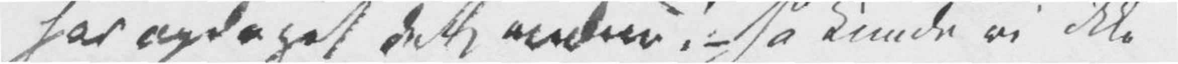har opdaget det endnu! – så kunde vi ikke
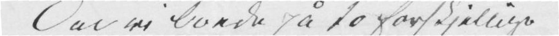Om vi boede på to forskjellige
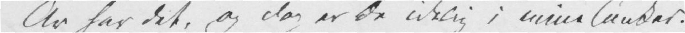År har det, og dog er De idelig i mine Tanker.
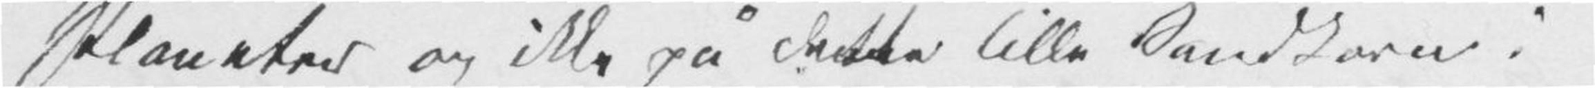Planeter og ikke på dette lille Sandkorn i
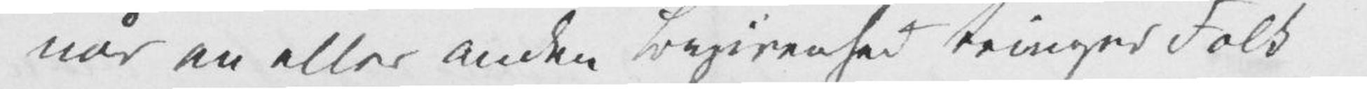når en eller anden Begivenhed tvinger Folk
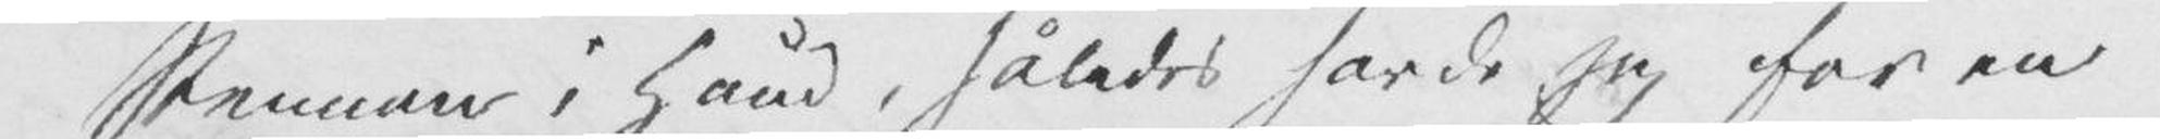Pennen i Hånd, således havde jeg for en
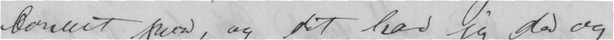Concert paa, og det har jeg da og
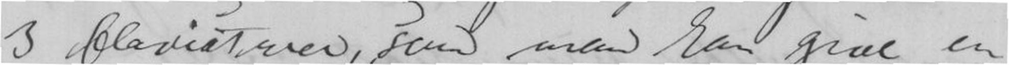3 Claviasturer, som man kan give en
